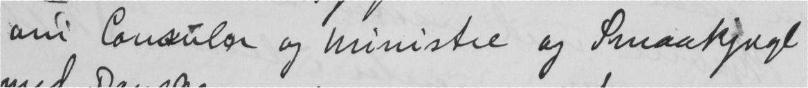om Consular og ministre og Smaakjagl
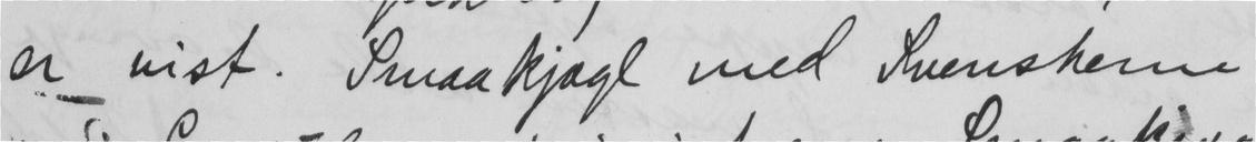er vist. Smaakjagl med Svenskerne
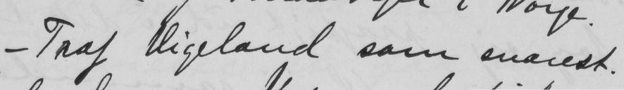- Traf Vigeland som snarest.
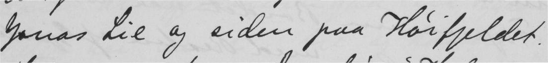Jonas Lie og siden paa Høifjeldet.
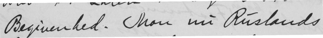Begivenbed. Mon nu Ruslands
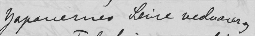Japanernes Leire vedvarer og
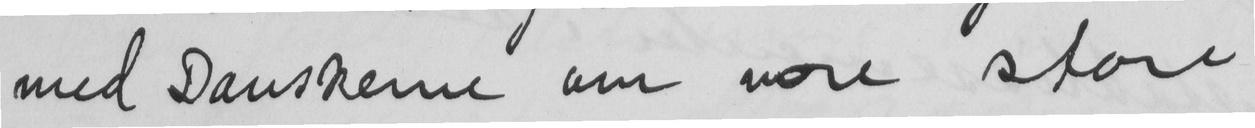med Danskerne om vore store
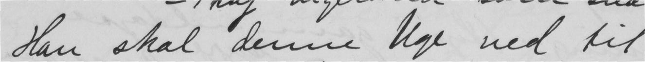Han skal denne Uge ned til
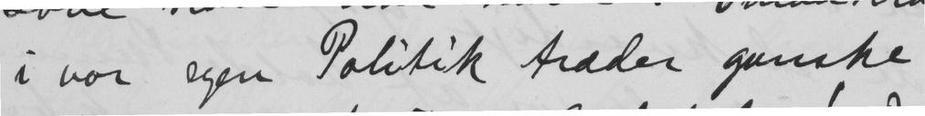i vor egen Politik træder ganske
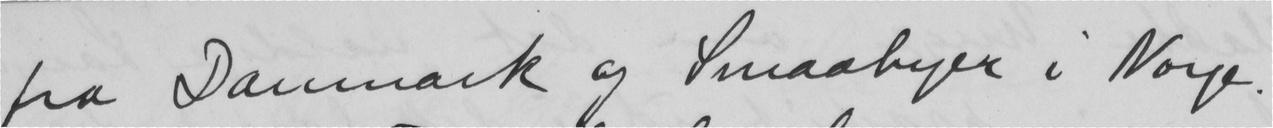fra Danmark og Smaabyer i Norge.
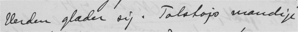Verden glæder sig . Tolstojs mandige
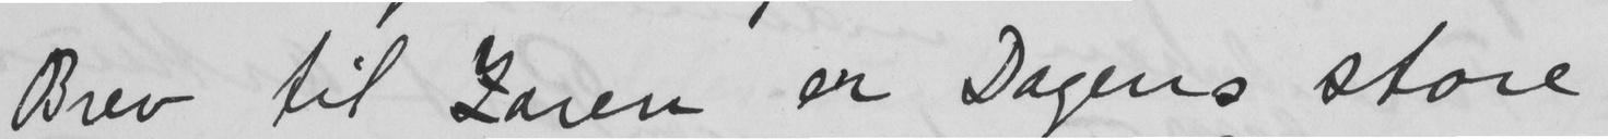Brev til Zaren er Dagens store
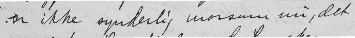er ikke synderlig morsom nu, det
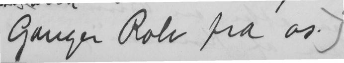Ganger Rolv fra os.
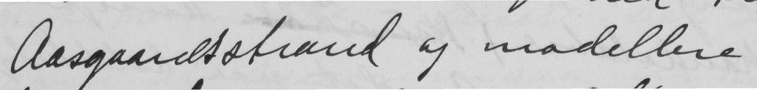Aasgaardsstrand og modellere
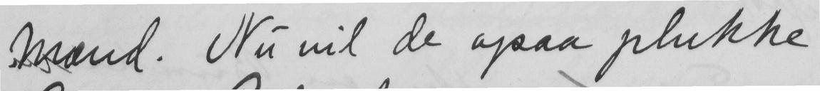Mand. Nu vil de ogsaa plukke
